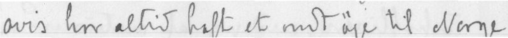avis har altid haft et ondt øje til Norge,
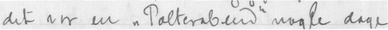det var en Polterabend nogle dage
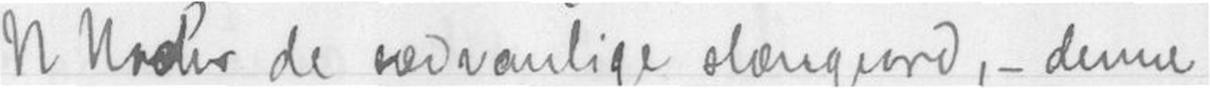N Noder de sædvanlige slængeord,- denne
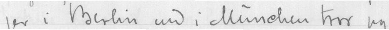jer i Berlin end i München tror jeg
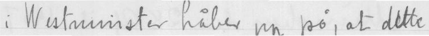i Westminister håber jeg på, at dette
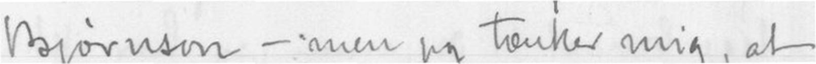Bjørnson - men jeg tænker mig, at
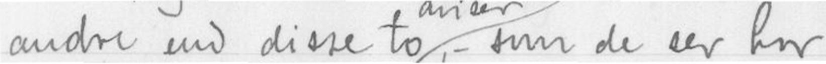andre end disse to,- som de ser har
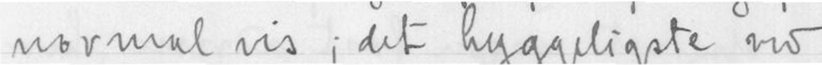normalt vis, det hyggeligste ved
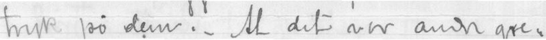tryk på dem,- At det var andre gre-
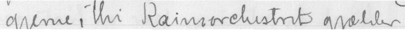gjerne, thi Raimorchesteret gjælder
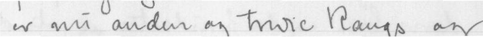er nu anden og tredje Rangs og
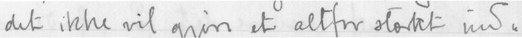det ikke vil gjøre et altfor sterkt ind-
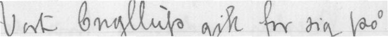Vort bryllup gik for sig på
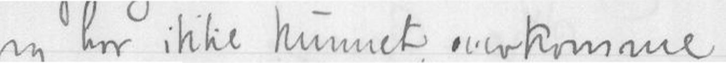Jeg har ikke kunnet overkomme
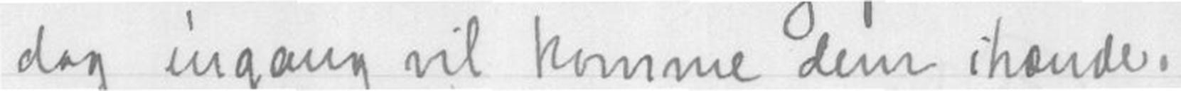dog éngang vil komme dem ihende.
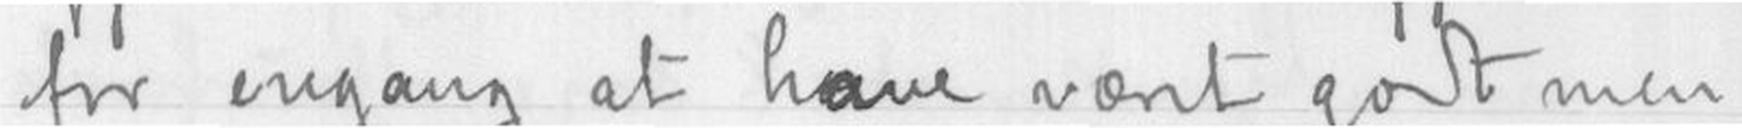for engang at have vært godt men
